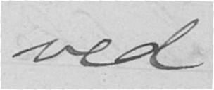ved
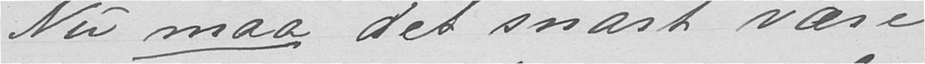Nu maa det snart være
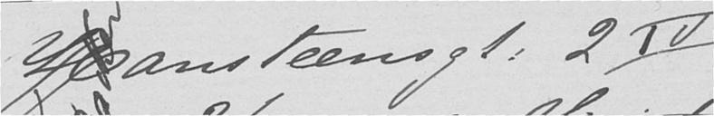Hansteensgt. 2 IV
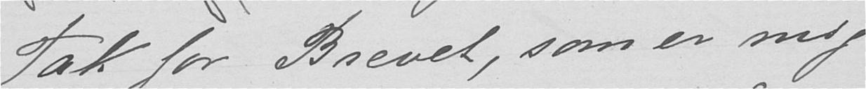Tak for Brevet, som er mig
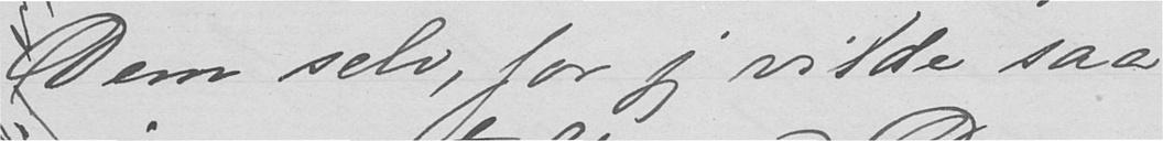Dem selv, for jeg vilde saa
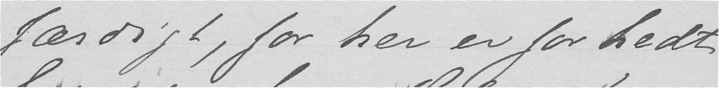færdigt, for her er for hedt
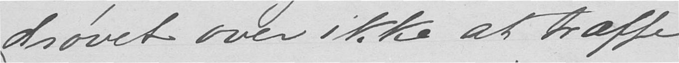drøvet over ikke at træffe
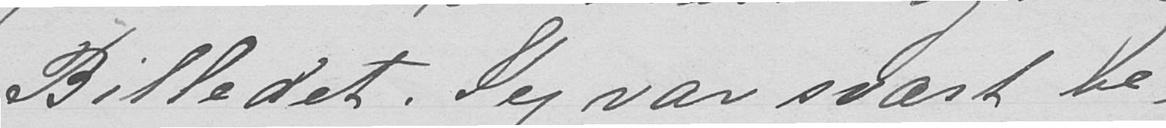Billedet. Jeg var svært be-
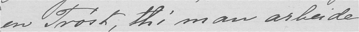en Trøst, thi man arbeider
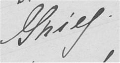Grieg.
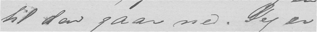til den gaar ned. Jeg er
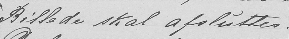Billede skal afsluttes.
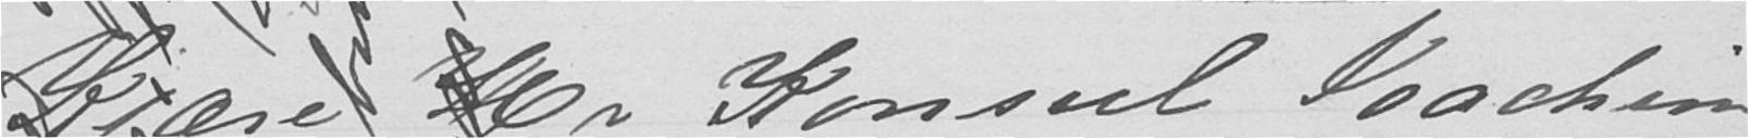Kjære Hr Konsul Joachim
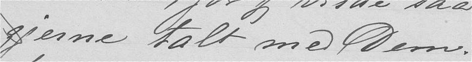gjerne talt med Dem.
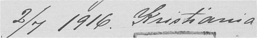2/7 1916. Kristiania.
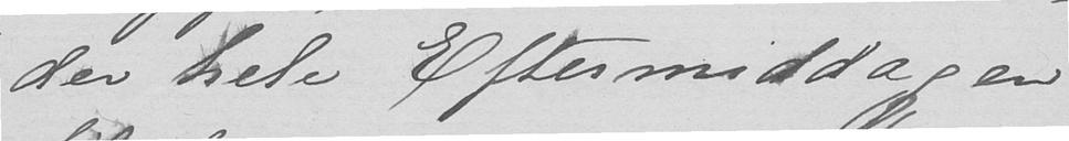der hele Eftermiddagen
